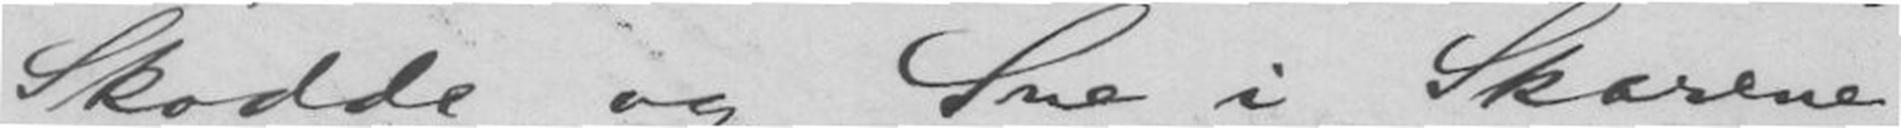Skodde og Sne i Skarene
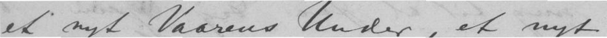et nyt Vaarens Under, et nyt
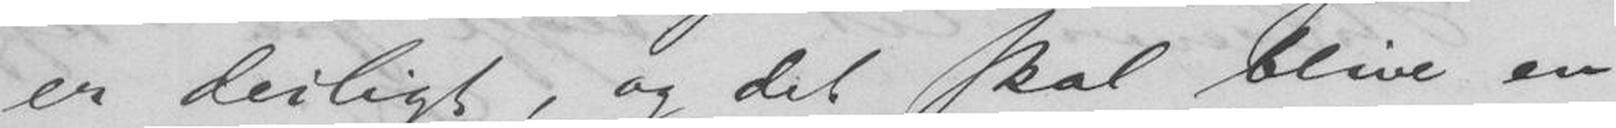er deiligt, og det skal blive en
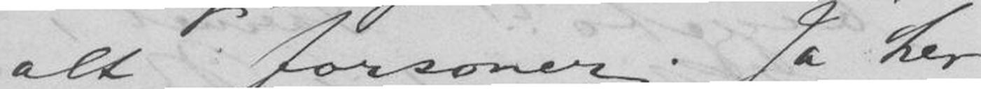alt forsoner. Ja her
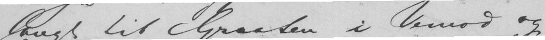langt til Graaten i Vemod og
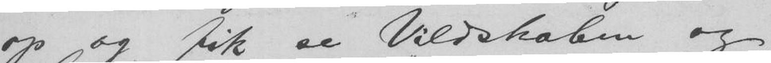op og fik se Vildskaben og
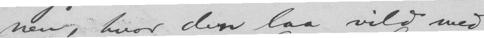nen, hvor den laa vild med
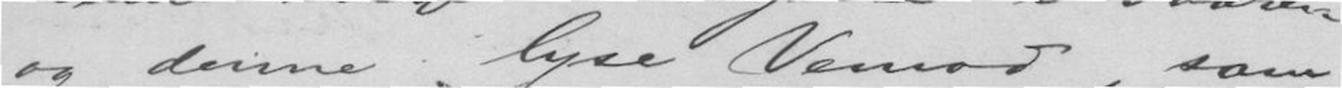og denne lyse Vemod, som
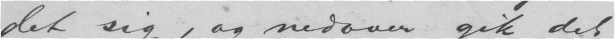det sig, og nedover gik det
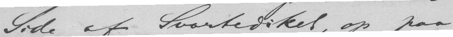Side af Svartediket, op paa
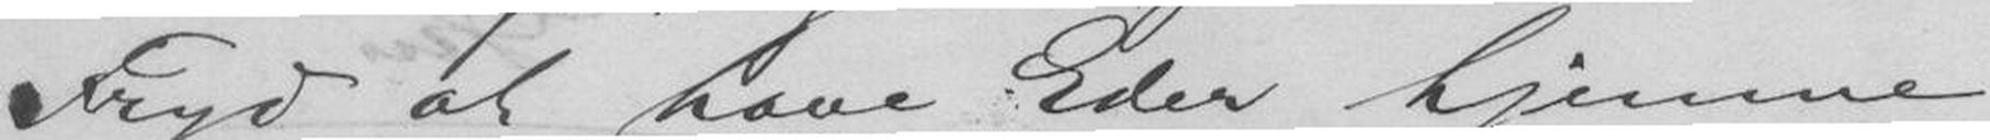Fryd at have Eder hjemme
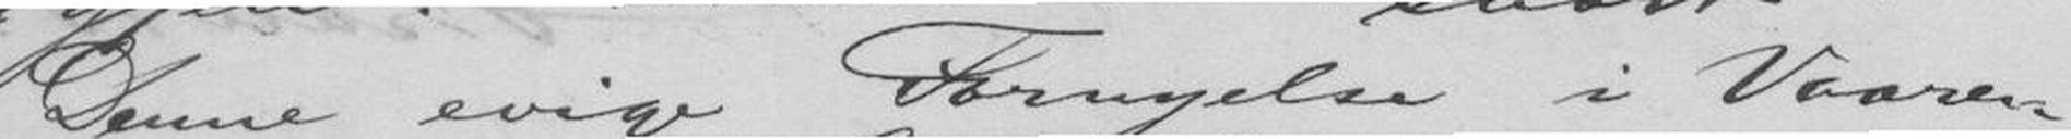Denne evige Fornyelse i Vaaren
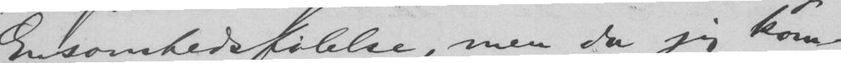Ensomhedsfølelse, men da jeg kom
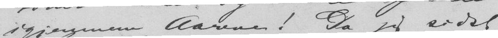igjennem Aarene! Da jeg sidst
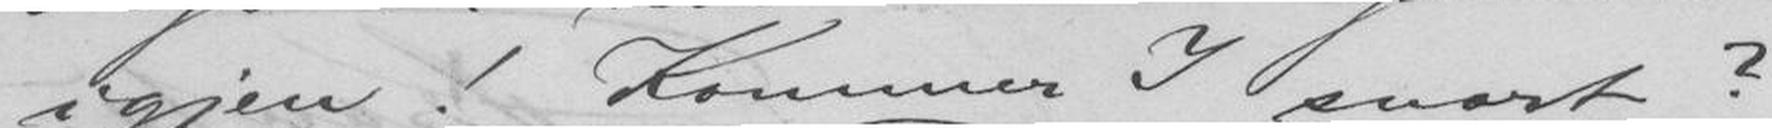igjen! Kommer I snart?
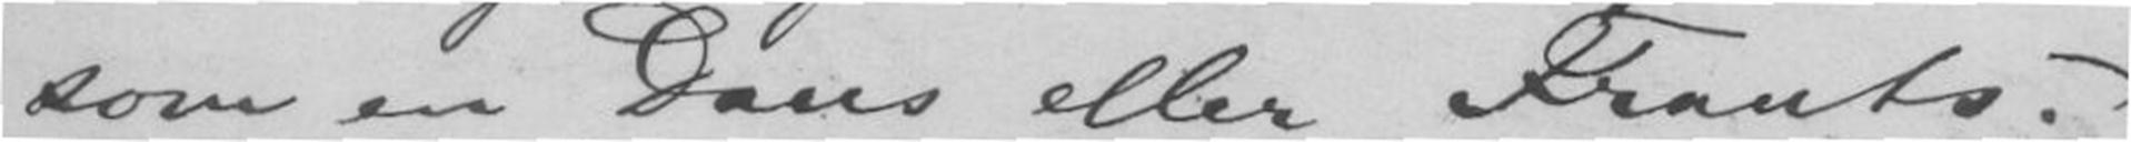som en Dans eller Frants.
